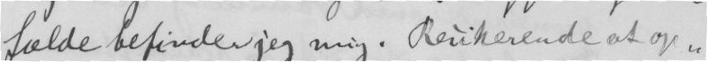fælde befinder jeg mig. Resikerende at op-
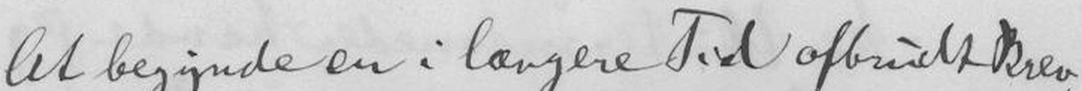At begynde en i længre Tid Afbrudt Brev-
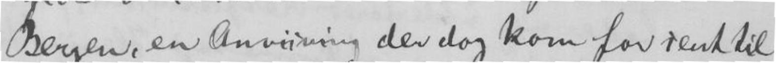Bergen, en Anvisning der dog kom for sent til
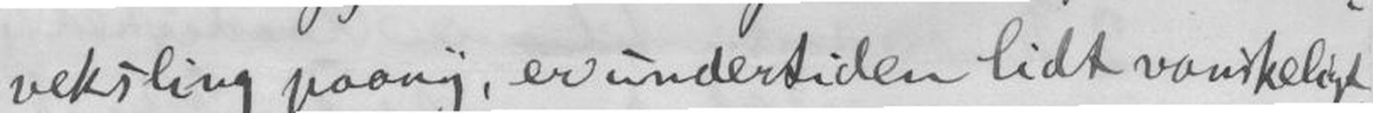veksling paany, er undertiden lidt vanskeligt,
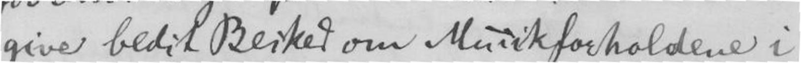give bedst Besked om Musikforholderne i
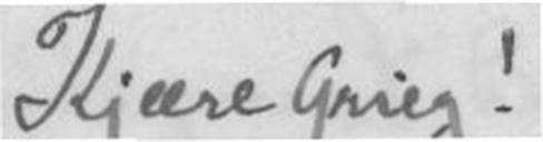Kjære Grieg!
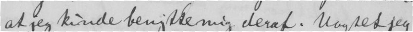at jeg kunde benytte mig deraf. Uagtet jeg
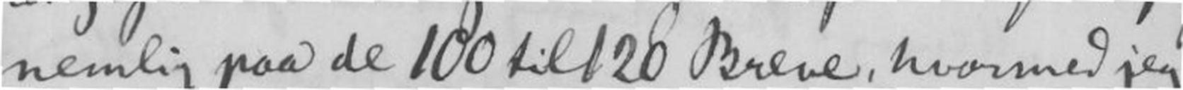nemlig paa de 100 til 120 Breve, hvormed jeg
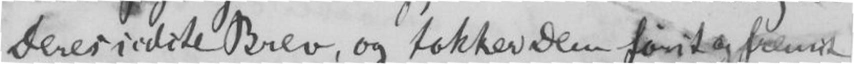Deres sidste Brev, og takker Dem først og fremst
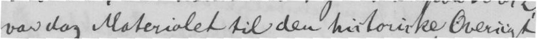var dog Materialet til den historiske Oversigt
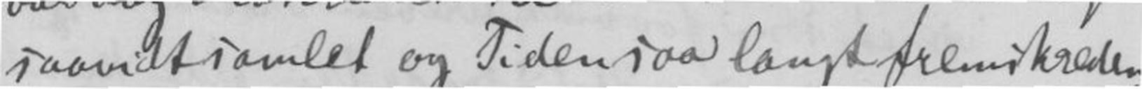saavidt samlet og Tiden saa langt fremskreden.
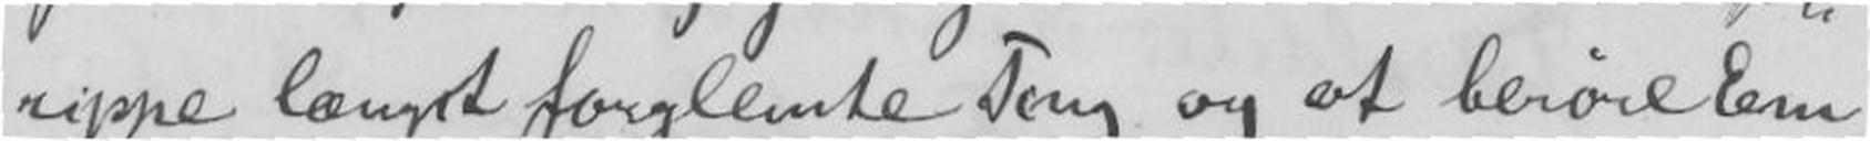rippe længst forglemte Ting og at berøre Em-
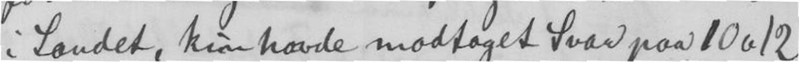i Landet, kun havde modtaget Svar paa 10 a 12
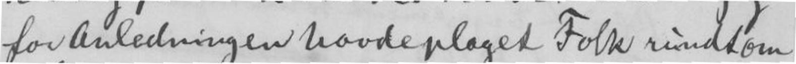for Anledningen havde plaget Folk rundt om
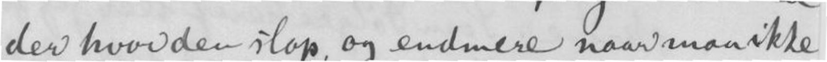der hvor den slap, og end mere naar man ikke
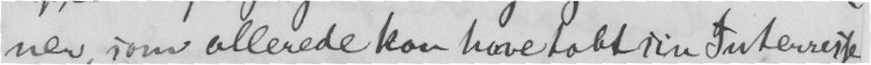ner, som allerede kan have tabt sin Interresse,
